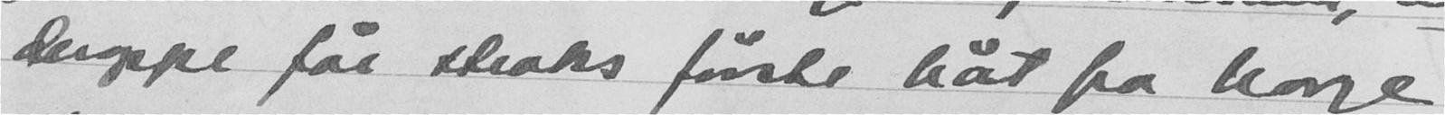deroppe får straks første båt fra Norge
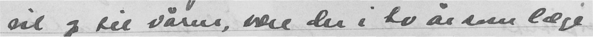vil og til våren, være der i to år som læge
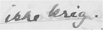ikke krig.
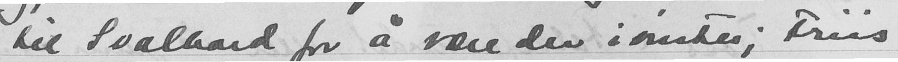til Svalbard for å være der i vinter; Friis
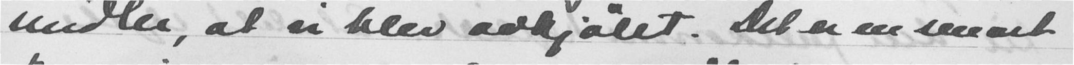midler, at vi blev avkjølet. Det er en smart
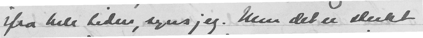ifra hele tiden, syns jeg. Men det er sterkt
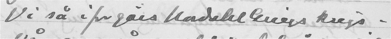Vi så i forgårs Nordahl Griegs krigs -
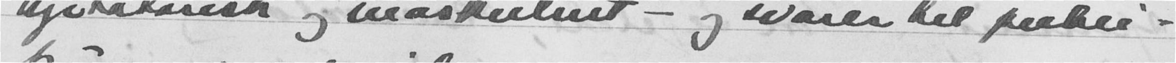agitatarisk og maskulint - og svarer til publi-
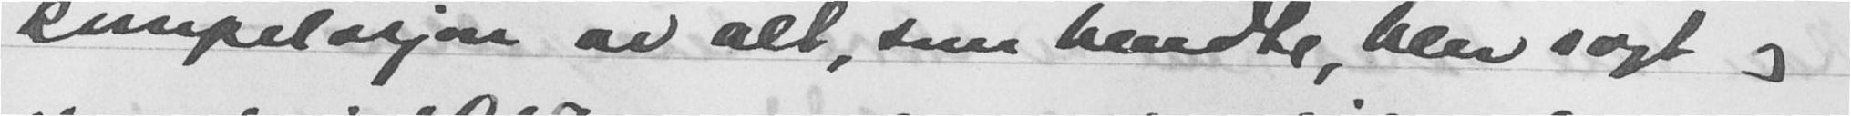kompilasjon av alt, som hendte, blev sagt og
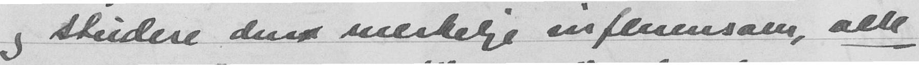og studere den merkelige influensaen, alle
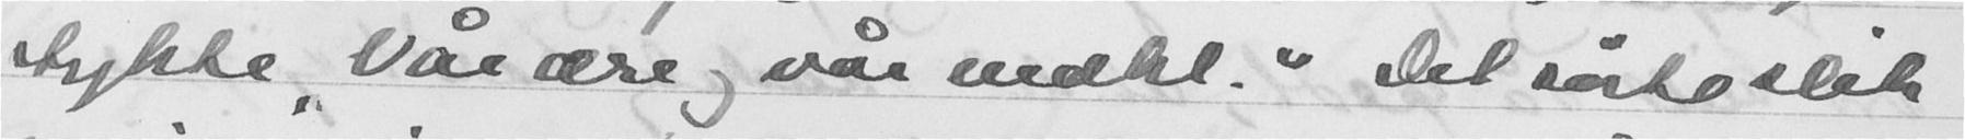stykke "Vår ære og vår makt." Det rørte slik
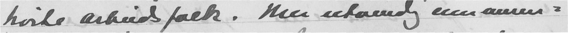hvite arbeidsfolk. Mer utvendig enn innen-
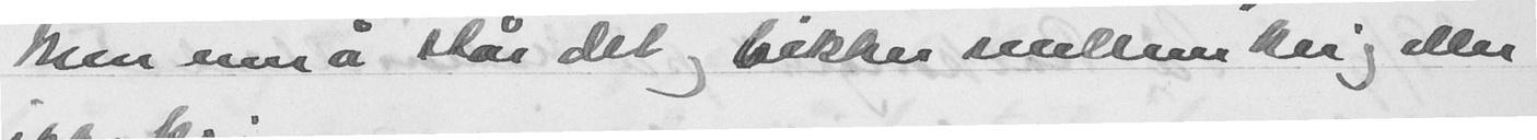Men ennå står det og bikker mellom krig eller
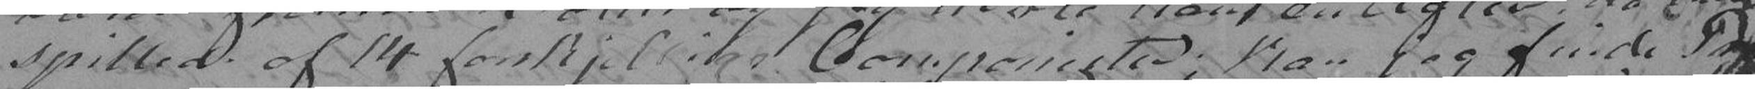spillede af 14 forskjellige Componister; kan jeg finde Pro-
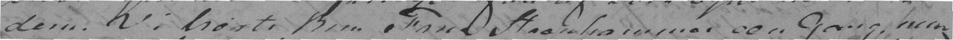dem. Vi hørte kun Frue Steenhammer een Gang, men
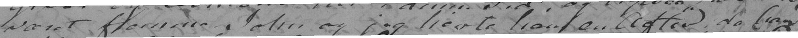været fremme John og jeg hørte han en Aften, da han
